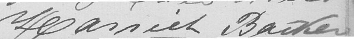Harriet Backer
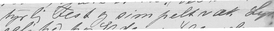tyrlig Fest og simpelt væk Lyk-
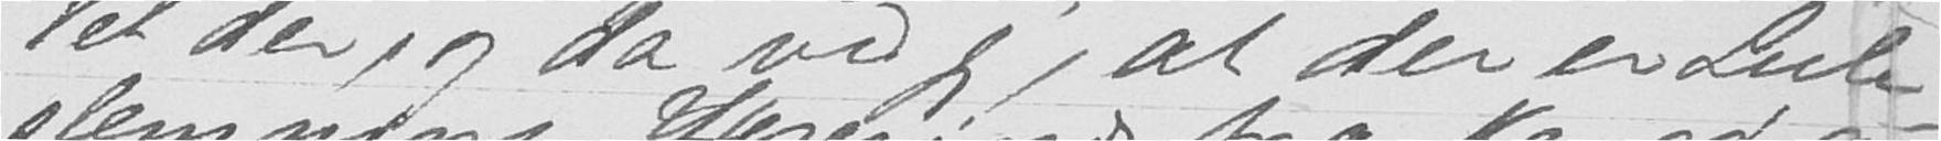let der, og da ved jeg, at der er Jule-
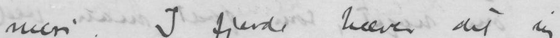meri. I fjerde hæver det sig
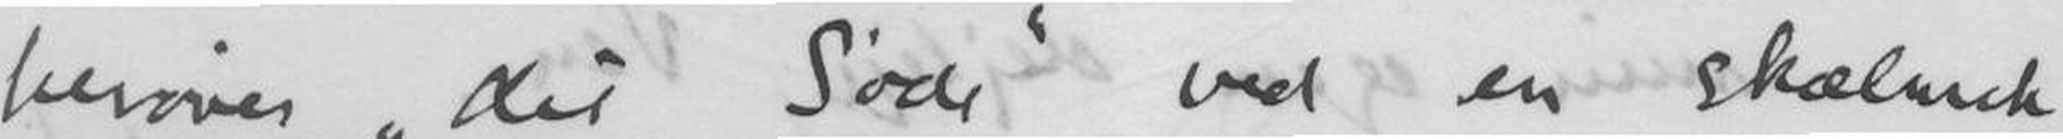berøves det søde ved en skælmsk
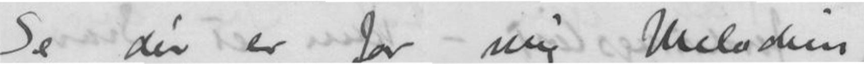Se dèr er for mig Melodien
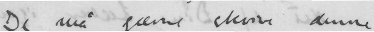De må gærne skrive denne
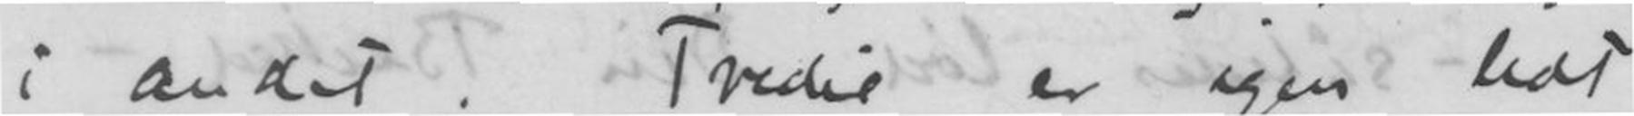i andet. Tredie er igen lidt
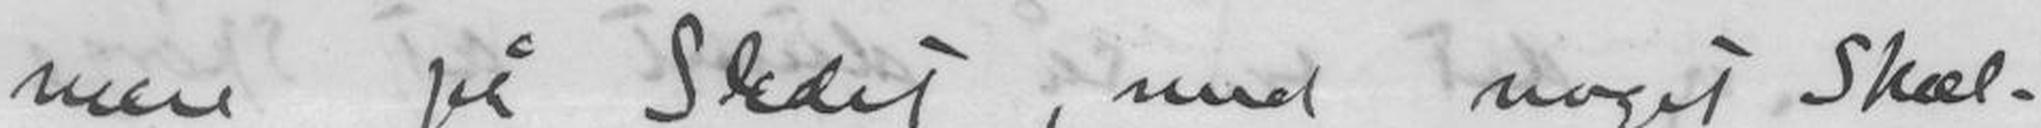mere på Stedet, med noget Skæl-
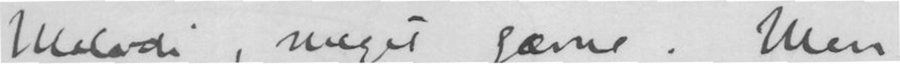Melodi, meget gærne. Men
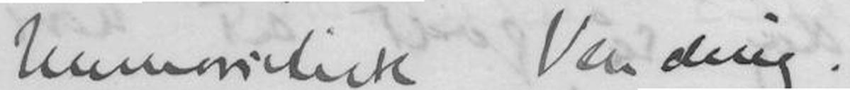humoristisk Vending.
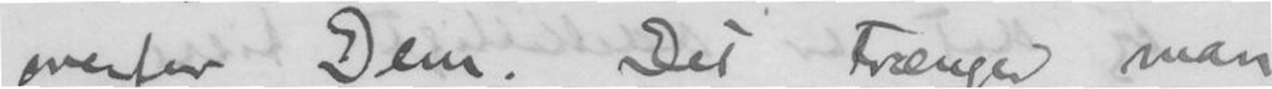overfor Dem. Det trænger man
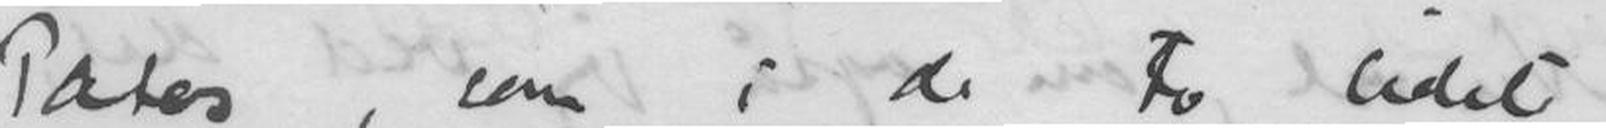Patos, som i de to lidet
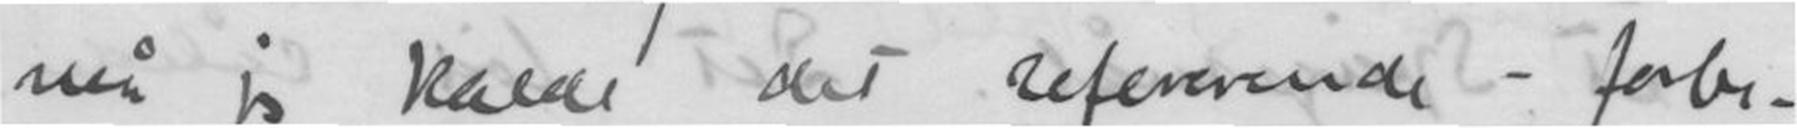må jeg kalde det refrerende - forbe-
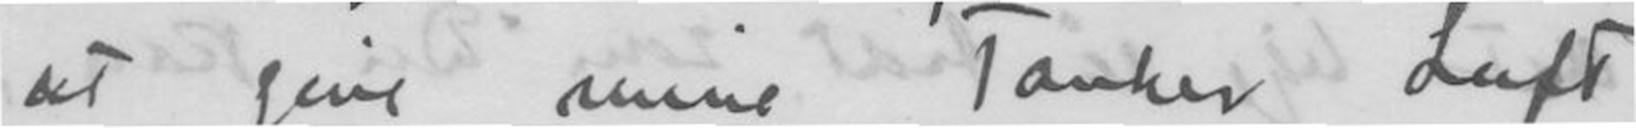at give mine Tanker Luft
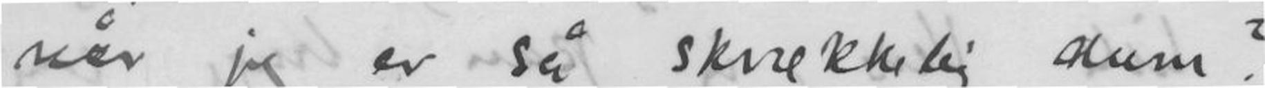Når jeg er så skrekkelig dum?
